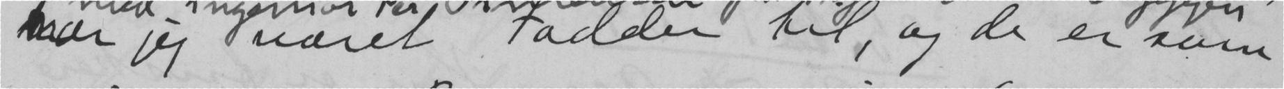har jeg været Fadder til, og de er som
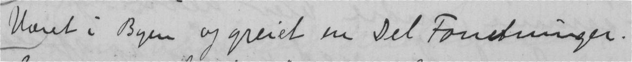Været i Byen og greiet en Del Forretninger.
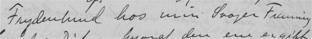Frydenlund hos min Svoger Frenning
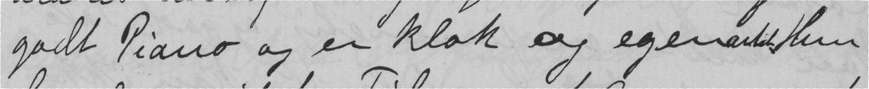godt Piano og er klok og egenartet. Hun
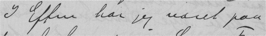I Eftm har jeg været paa
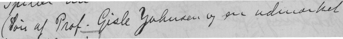(Søn af Prof. Gisle Johnsen og er udmærket
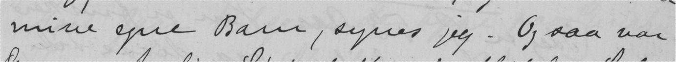mine egne Barn, synes jeg. Og saa var
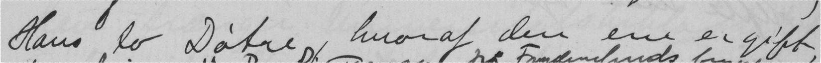Hans to Døtre, hvorat den ene er gift,
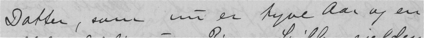Datter, som nu er tyve aar og en
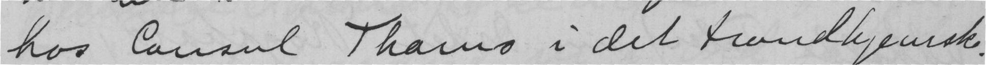hos Consul Thams i det trondhjemske.
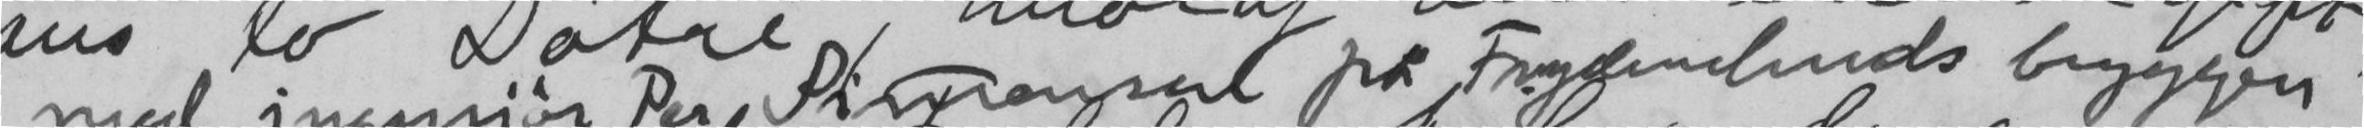med ingeniør Per Simensen (på Frydenlunds bryggeri
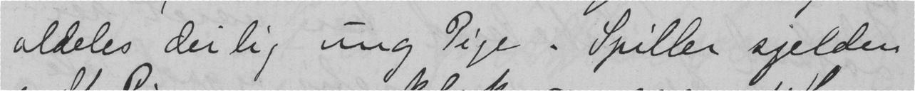aldeles deilig ung Pige. Spiller sjelden
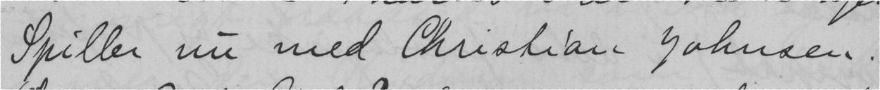Spiller nu med Christian Johnsen.
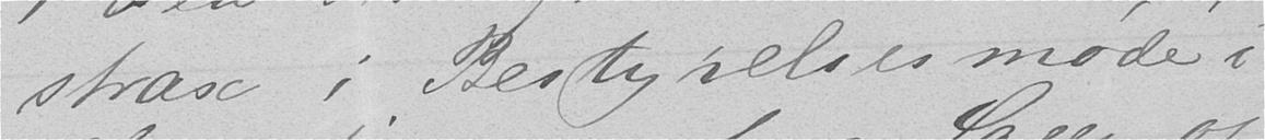strax i Bestyrelsesmøde i
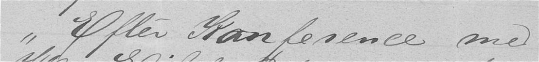"Efter Konference med
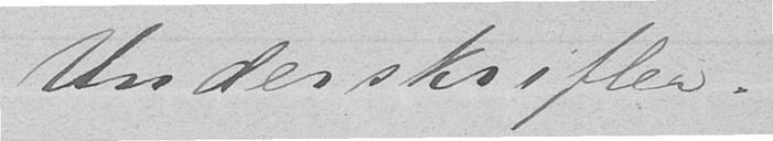Underskrifter.
